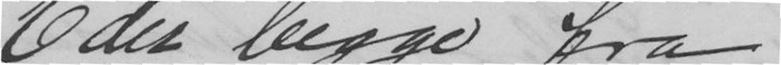Eder begge fra,
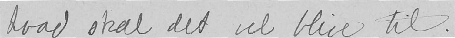hvad skal det vel blive til.
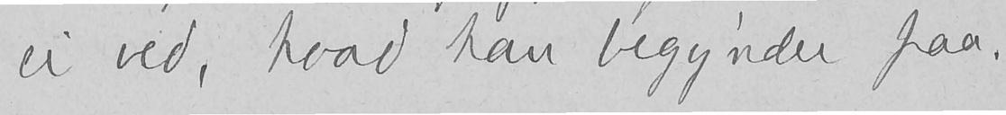ei ved, hvad han begynder paa.
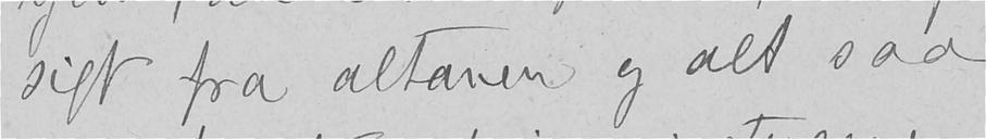sigt fra altanen og alt saa
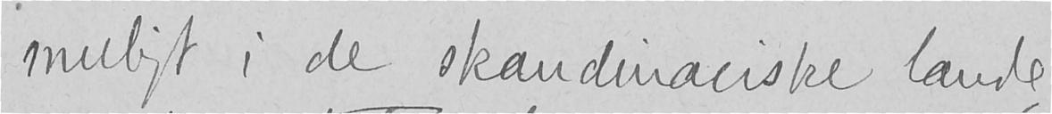muligt i de skandinaviske lande
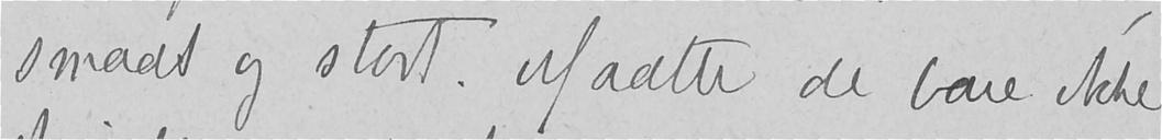smaat og stort. Maatte de bare ikke
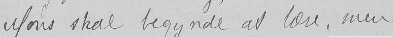Jons skal begynde at læse, men
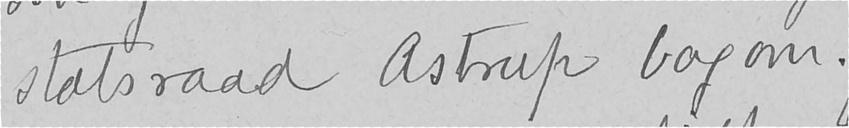statsraad Astrup bagom.
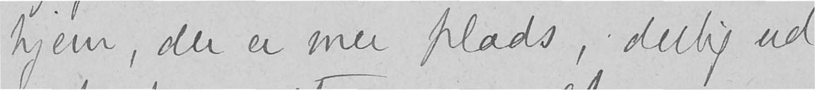hjem, der er mer plads, deilig ud-
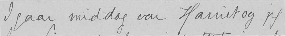Igaar middag var Harriet og jeg
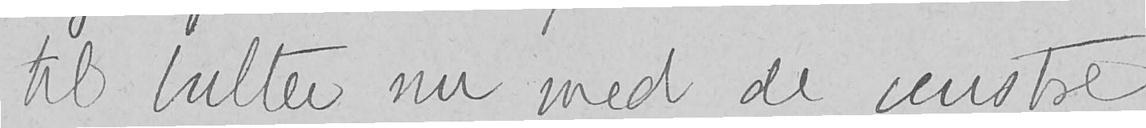til bulter nu med de venstre,
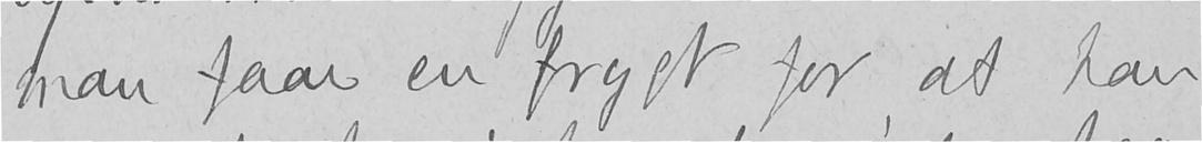man faar en frygt for at han
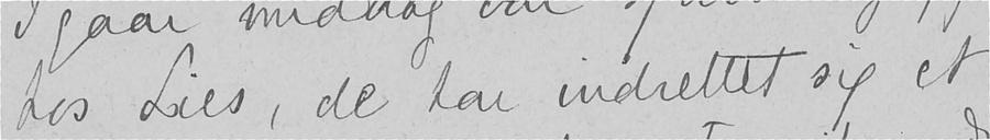hos Lies, de har indrettet sig et
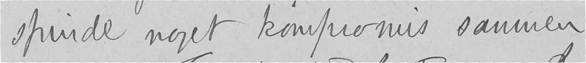spinde noget kompromis sammen
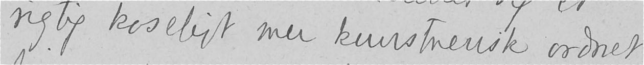rigtig koseligt mer kunstnerisk ordnet
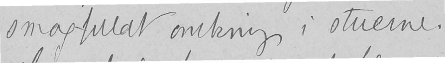smagfuldt omkring i stuerne.
